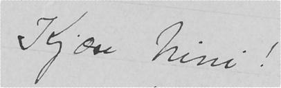Kjære Nini!
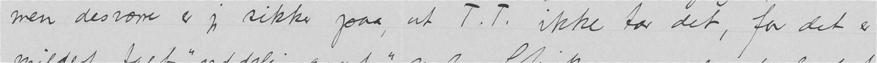men desv<æ>rre er jeg sikker paa, at T.T. ikke tar det, for det er
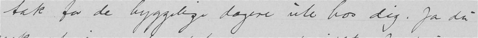tak for de hyggelige dagene ute hos dig. Ja du
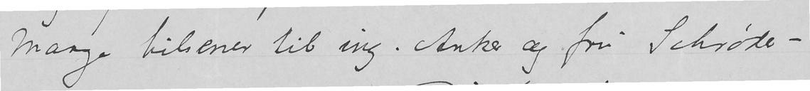Mange hilsener til ing. Anker og fru Schrøder-
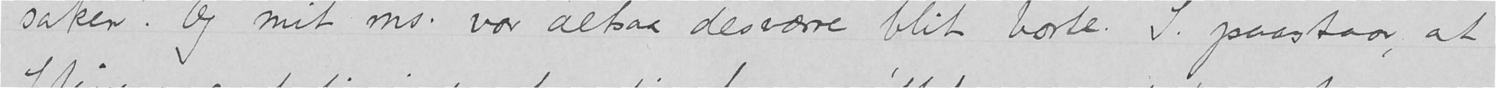saken». Og mit ms. var altsaa desv<æ>rre blit borte. S. paastaar at
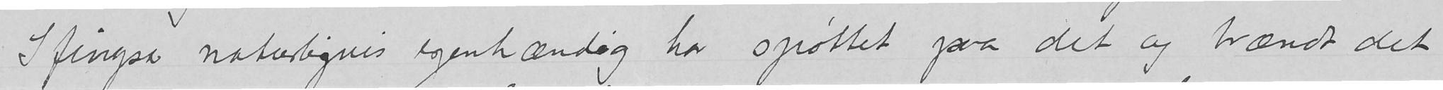Sfingsen naturligvis egenhændig har spøttet paa det og brændt det
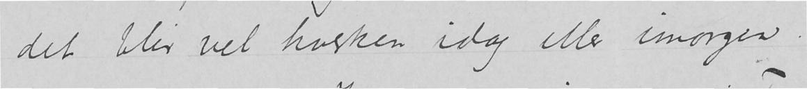det blir vel hverken idag eller imorgen.
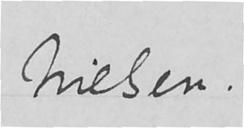Din hengivne
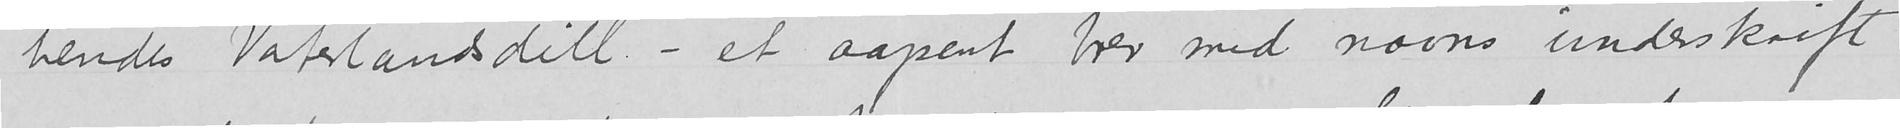hendes Vaterlandsdill – et aapent brev med navns indskrift
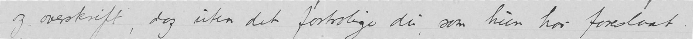og overskrift, dog uten det fortrolige du som hun har foreslaat.
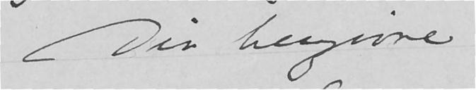Nielsen.
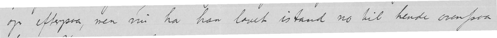op efterpaa, men nu har han lavet istand no til hende ovenpaa
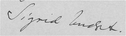Sigrid Undset.
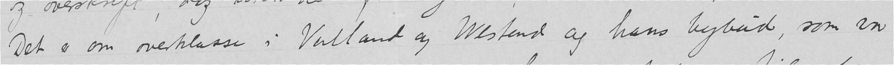Det er om overklasse i <Vestkant> og Westend og hans bybud, som var
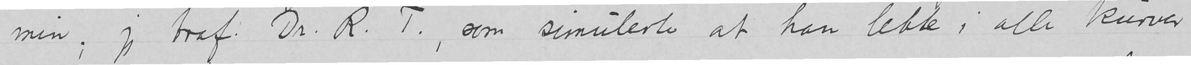min, jeg traf Dr. R.T., som simulerte at han lette i alle kurver
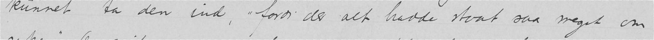kunnet ta den ind, «fordi der alt havde staat saa meget om
